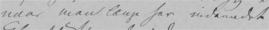naar man længe har indaandet
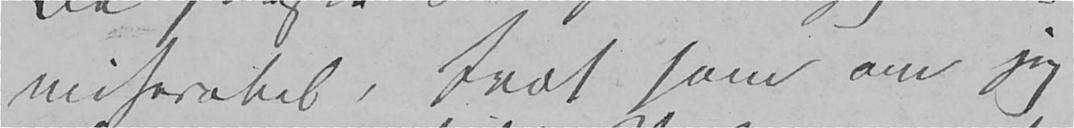miserabel, træt som om jeg
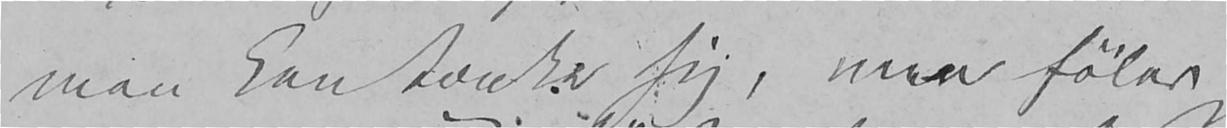man kan tænke sig, men føler
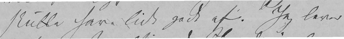skulle have lidt godt af. Jeg lever
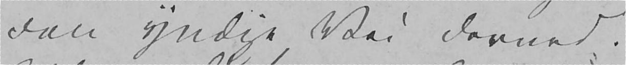den yndige Vei derned.
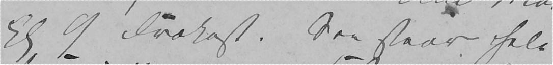Kl 9 Frokost. Saa staar hele
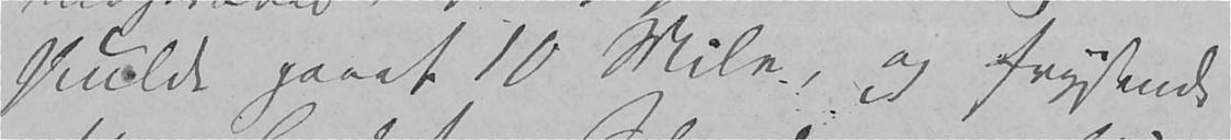skulde gaaet 10 Mile, og frysende
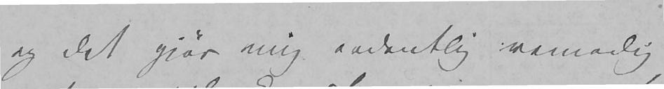og det gjør mig ordentlig vemodig
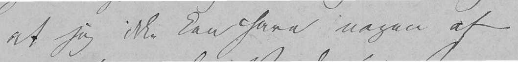at jeg ikke kan have nogen af
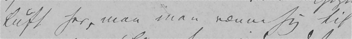Luft her, maa man vænne sig til
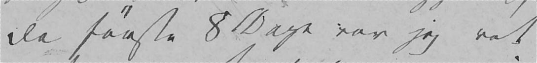De første 8 Dage var jeg ret
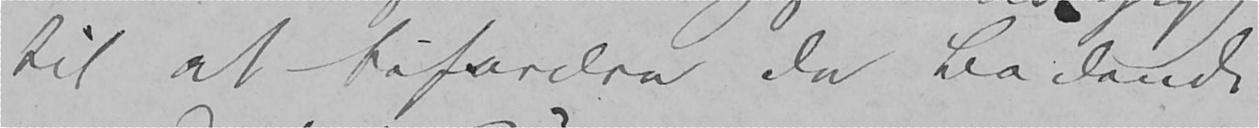til at befordre de Badende
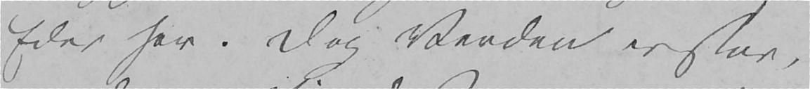Eder her. Dog Verden er stor,
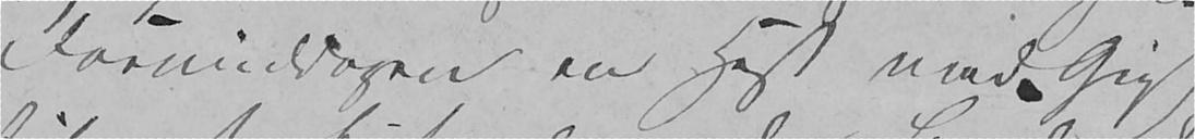Formiddagen en Hest med Gigh
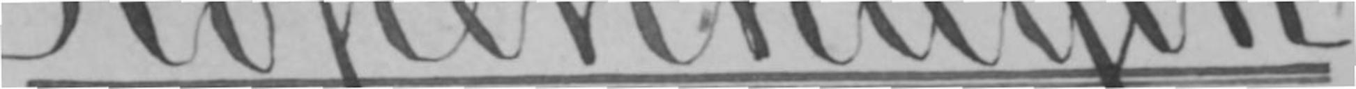Kopenhagen.
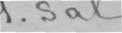1. Sal.
